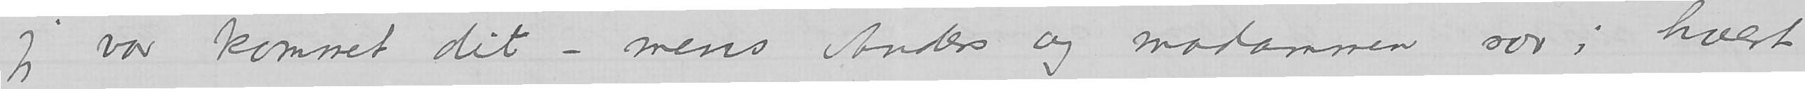jeg var kommet dit – mens Anders og madammen sov i hvert
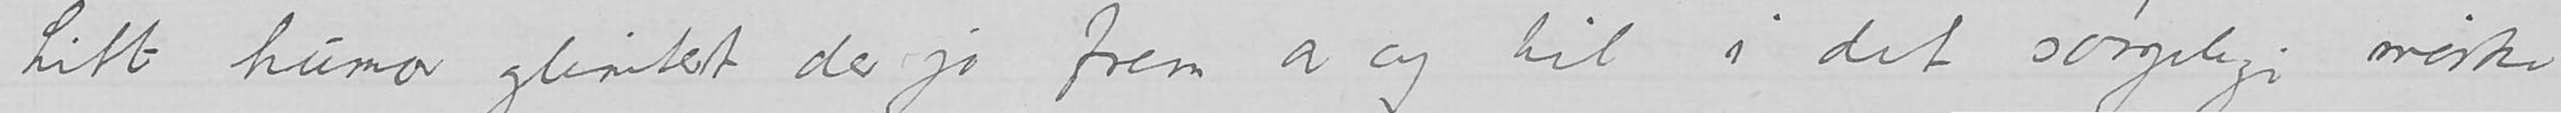Litt humor glimtet der jo frem av og til i det sørgelige mørke
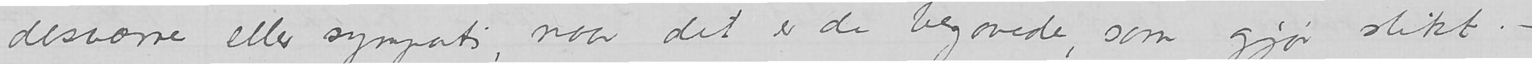desværre eller sympati, naar det er de begavede, som gjør slikt. –
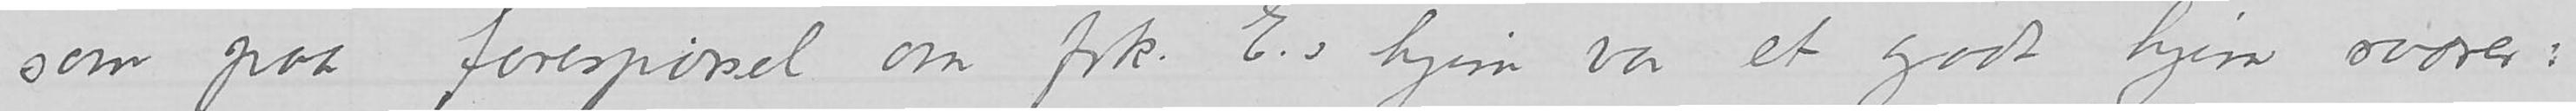–. som paa forespørsel om frk. E.s hjem var et godt hjem svarer:
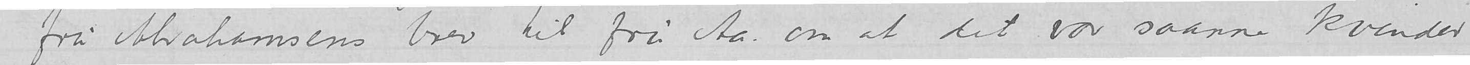– fru Abrahamsens brev til fru Aa. om at det var saanne kvinder
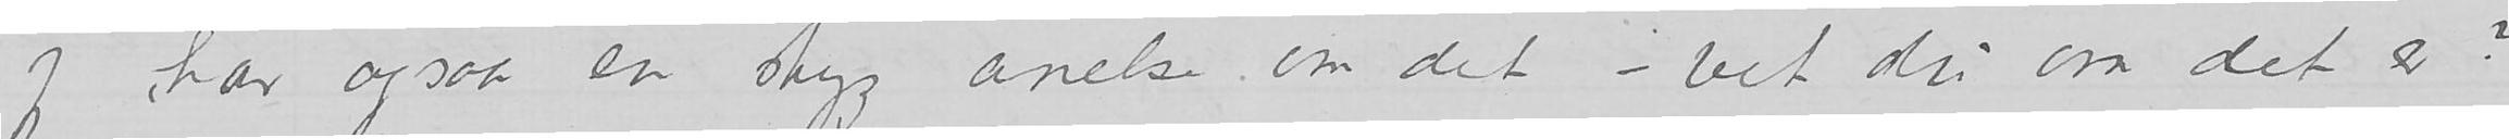jeg har ogsaa en styg anelse om det – vet du om det er?
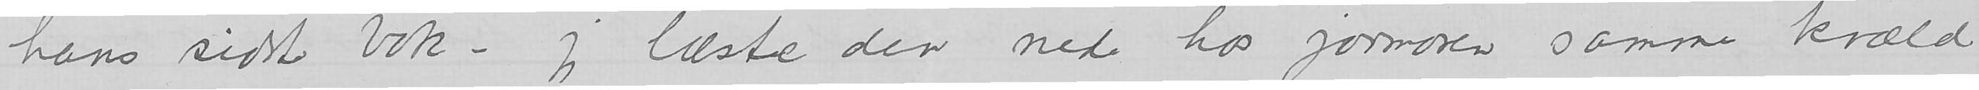hans sidste bok – jeg læste den nede hos jormoren samme kvæld
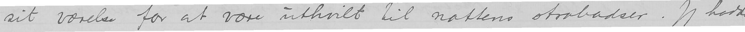sit værelse for at være uthvilt til nattens strabaser. Jeg hadde
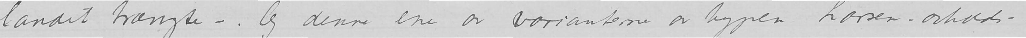landet trængte –. Og denne ene av varianterne av typen Larsen-avholds
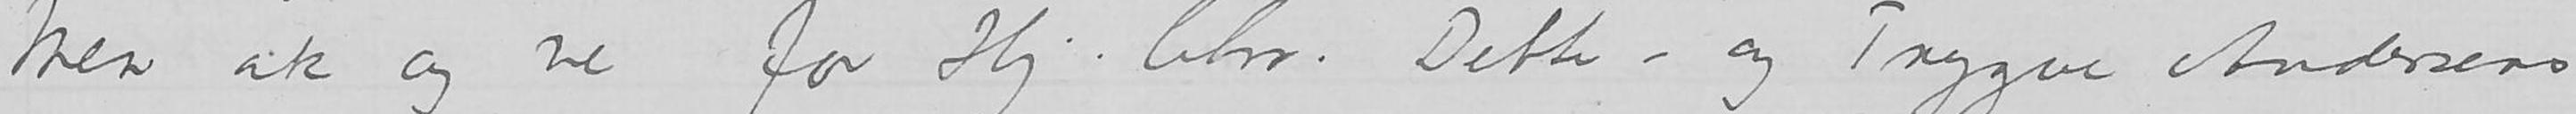Men ak og ve for Hj. Chr. Dette – og Trygve Andersens
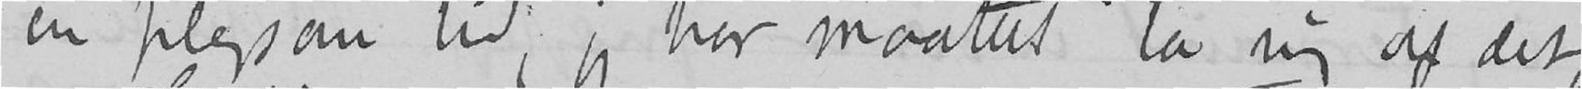en plagsom tid, jeg har maattet ta mig af det,
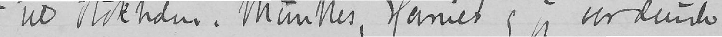til Stokholm. Munthe, Harriet og jeg var derude
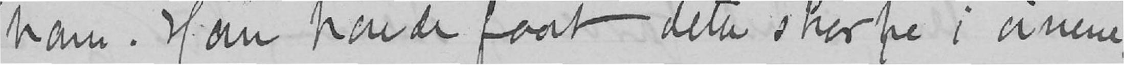ham. Han havde faat dette skarpe i øinene,
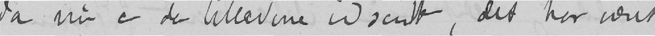Ja nu er da billedene udsendt, det har været
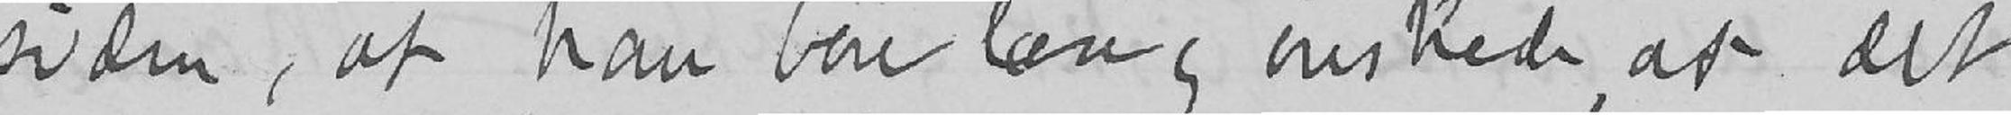siden, at han bare længe ønskede, at det
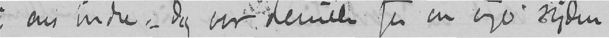i ens indre. Jeg var derude for en uge siden,
